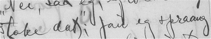take dat"; jau eg spraang
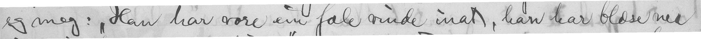eg meg: "Han har vore ein fale vinde inat, han har blæse nea
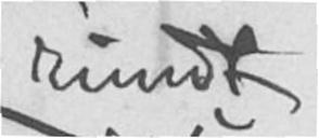rundt
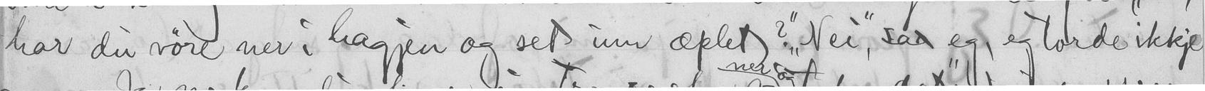har du vøre ner i hagjen og set um æplet?" "Nei", sa eg, eg torde ikkje
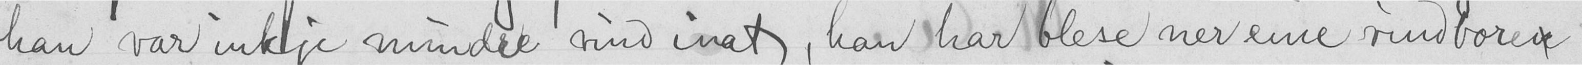han var inkje mindre vind inat, han har blese ner eine vindbore
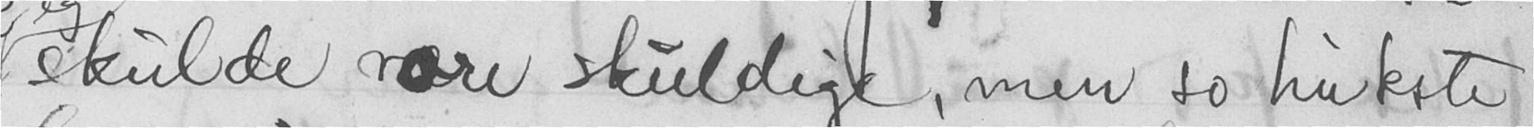skulde være skuldige, men so hukste
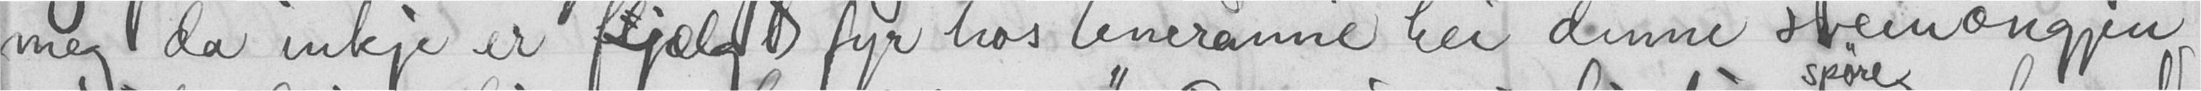meg da inkje er fjælgt fyr hos teneranne hei dinne sveinongjen
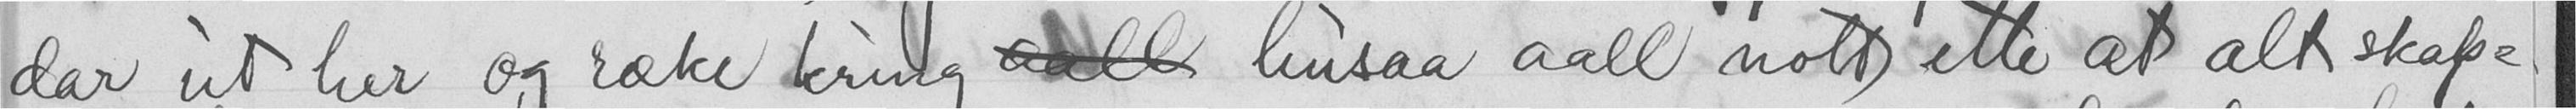dar ut her og ræke kring  husaa aall nott ette at alt skape-
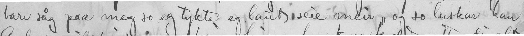bare såg paa meg so eg tykte og laut seie meir "og so luskar han
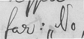far: "No,
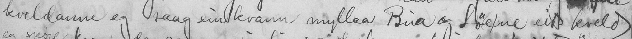kveldanne eg saag ein kvann myllaa Bua og Søene eit kveld
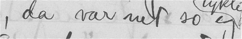, da var net so eg
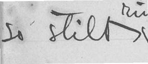so stilt
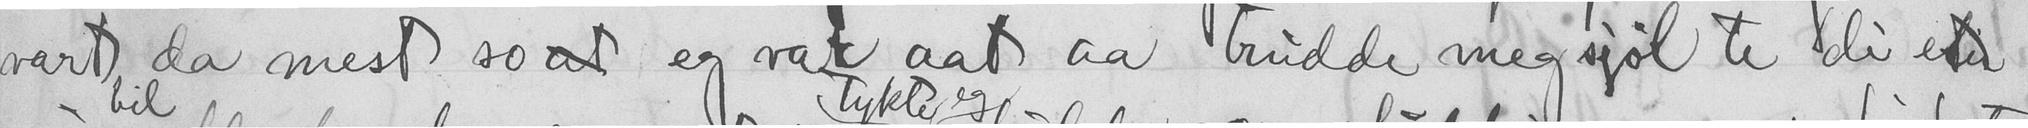vart da mest so at eg var aat aa trudde meg sjøl te di etu
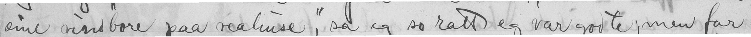eine vindbore paa veahuse", sa eg so ratt eg var god te, men far
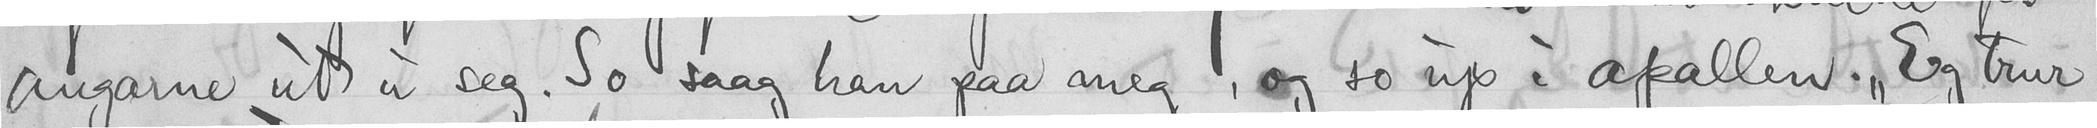augarne ut u seg. So saag han paa meg, og so up i apallen. "Eg trur
